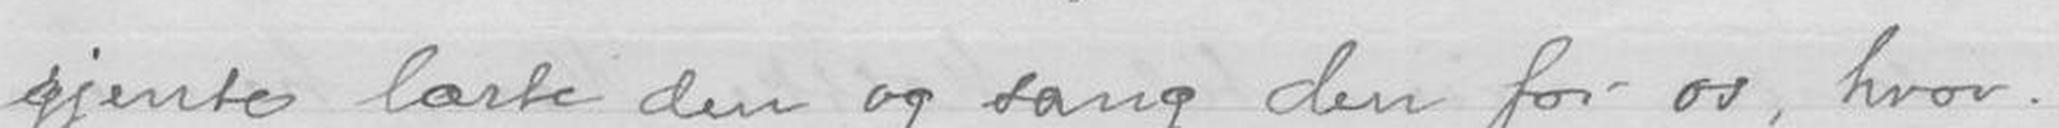gjente lærte den og sang den for os, hvor
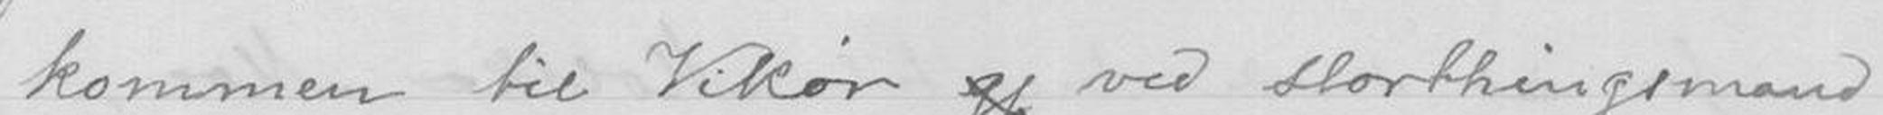kommen til Vikør ved storthingsmand
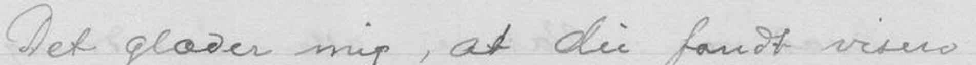Det glæder mig, at du fandt visen
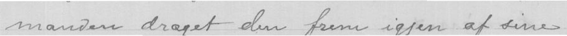manden draget den frem igjen af sine
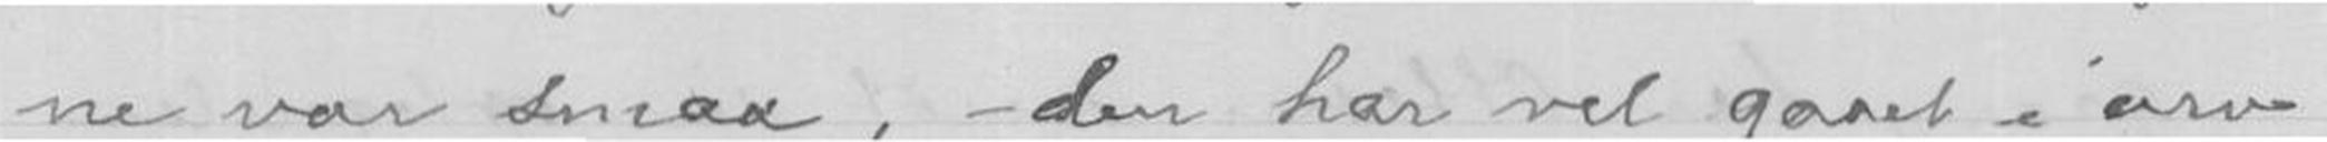ne var smaa, - den har vel gaaet i arv
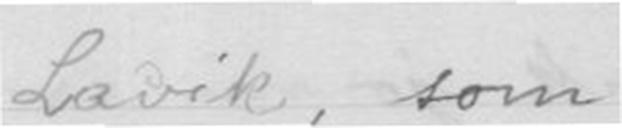Lavik, som
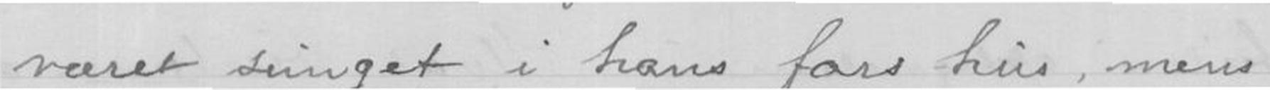været sunget i hans hus, mens
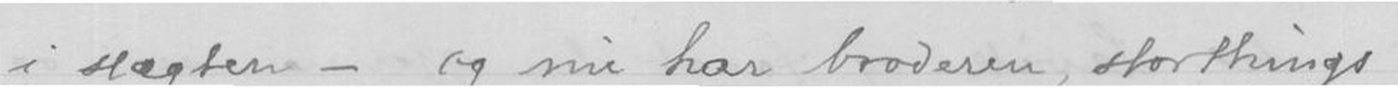i slægten - og nu har broderen, storthings-
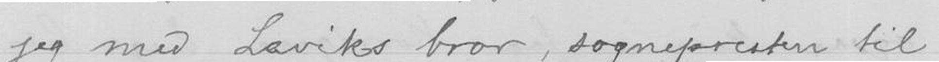jeg med Laviks bror, sognepresten til
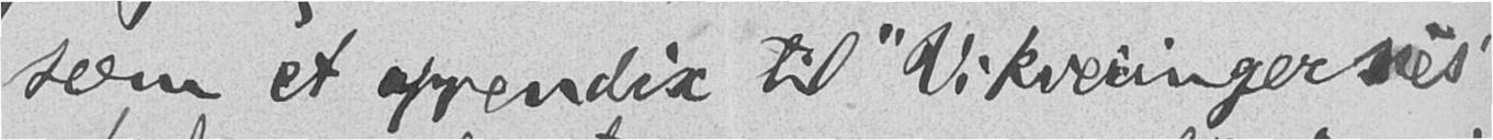som et appendix til "Vikveiringernes"
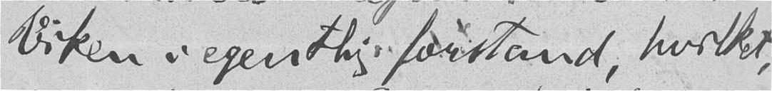Viken i egentlig forstand, hvilket,
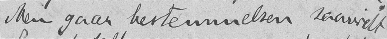Men gaar bestemmelsen saavidt
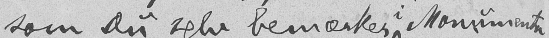som Du selv bemærker i Monumenta
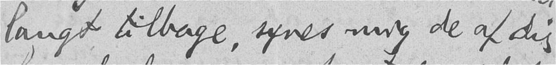langt tilbage, synes mig de af Dig
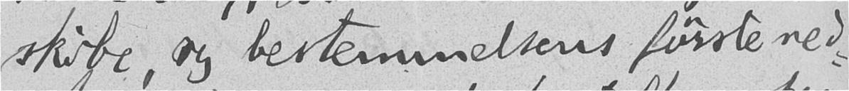skibe, og bestemmelsens første ned-
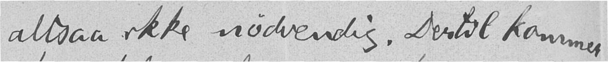altsaa ikke nødvendig. Dertil kommer,
